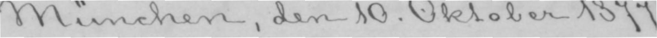München, den 10. Oktober 1877.
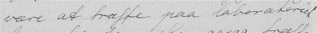være at træffe paa laboratoriet
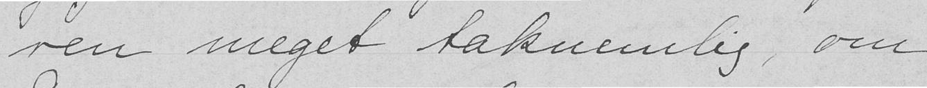ren meget taknemlig, om
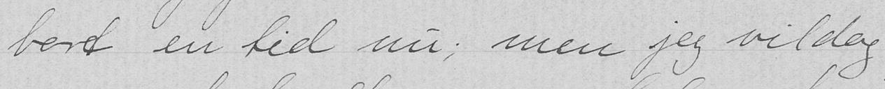bort en tid nu; men jeg vil dog
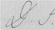D.S.
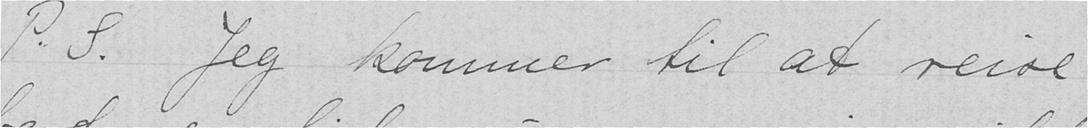P.S. Jeg kommer til at reise
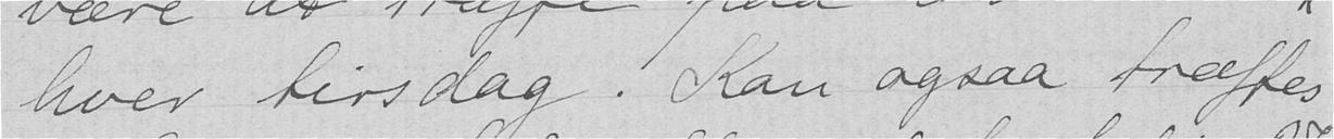hver tirsdag. Kan ogsaa træffes
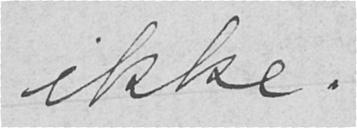ikke.
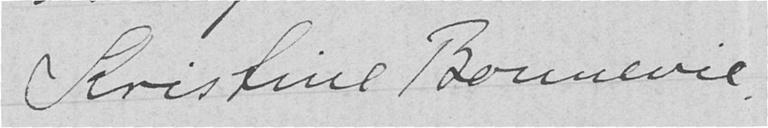Kristine Bonnevie.
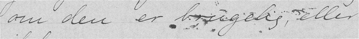om den er brugelig, eller
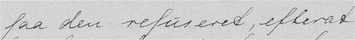faa den refuseret, efterat
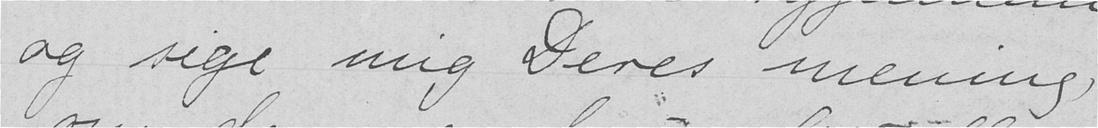og sige mig Deres mening,
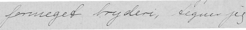formeget bryderi, tegner jeg
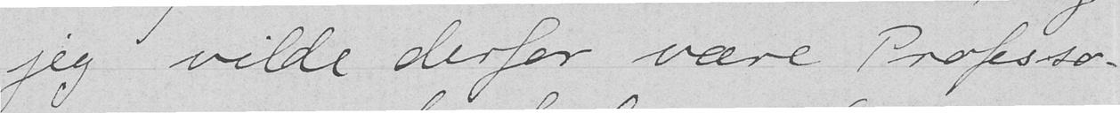jeg vilde derfor være Professo-
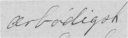ærbødigst
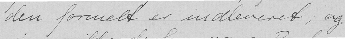den formelt er indleveret; og
